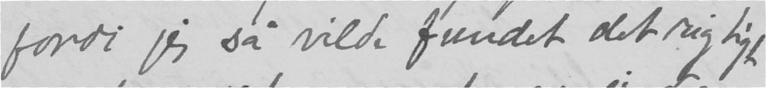fordi jeg så vilde fundet det rigtigt
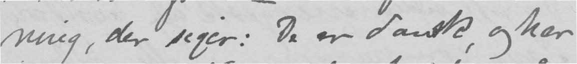ning, der siger: De er dansk, og har
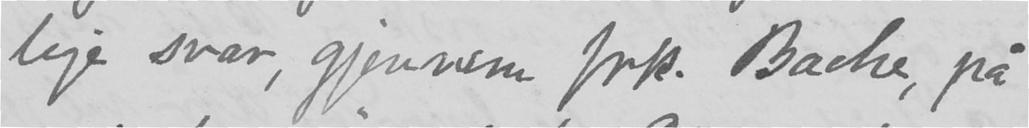lige svar, gjennem frk. Bache, på
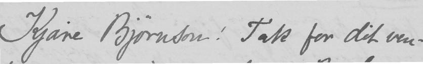Kjære Bjørnson! Tak for dit ven-
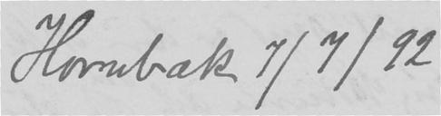Hornbæk 7/7/92
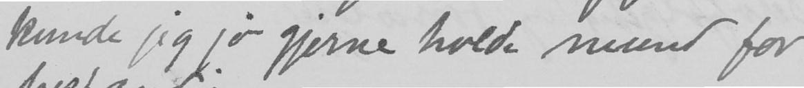kunde jeg jo gjerne holde mund for
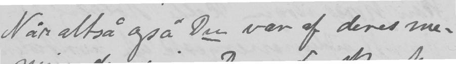Når altså også Du var af deres me-
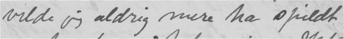vilde jeg aldrig mere ha spildt
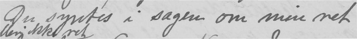Du syntes i sagen om min ret
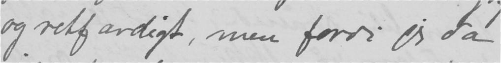og retfærdigt, men fordi jeg da
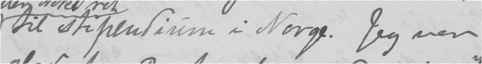til stipendium i Norge. Jeg var
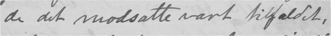de det modsatte vært tilfældet,
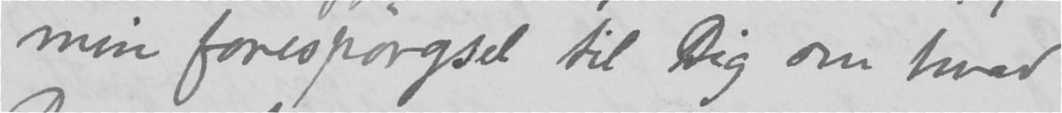min forespørgsel til Dig om hvad
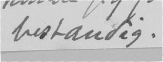bestandig.
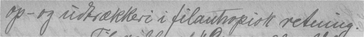op- og udtrækkeri i filantropisk retning.
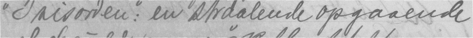"Isisorden:" en straalende opgaaende
